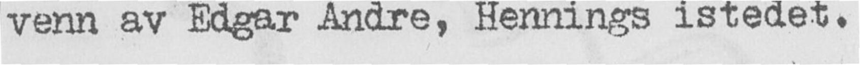venn av Edgar Andre, Hennings istedet.
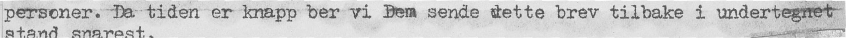personer. Da tiden er knapp ber vi Dem sende dette brev tilbake i undertegnet
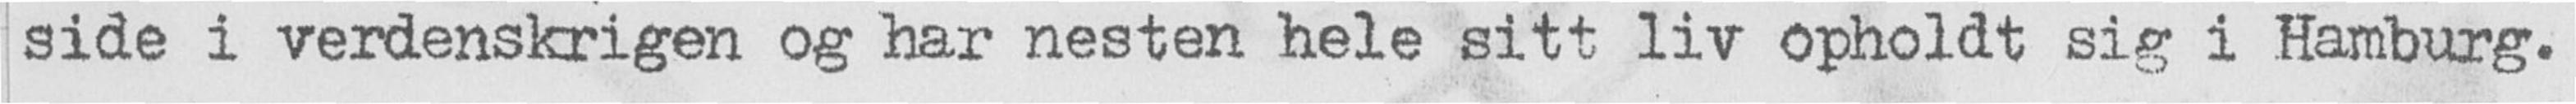side i verdenskrigen og har nesten hele sitt liv opholdt sig i Hamburg.
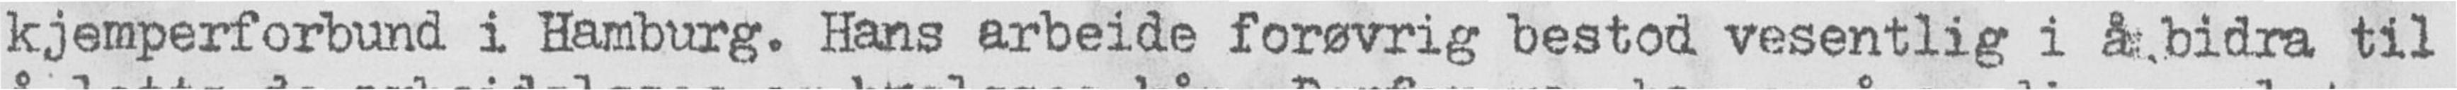kjemperforbund i Hamburg. Hans arbeide forøvrig bestod vesentlig i å bidra til
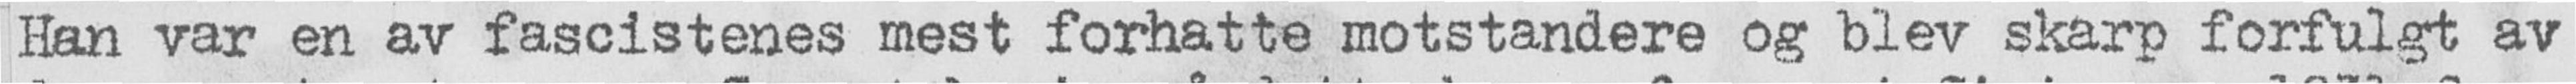Han var en av fascistenes mest forhatte motstandere og blev skarp forfulgt av
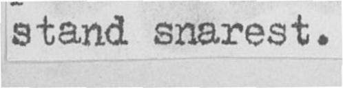stand snarest.
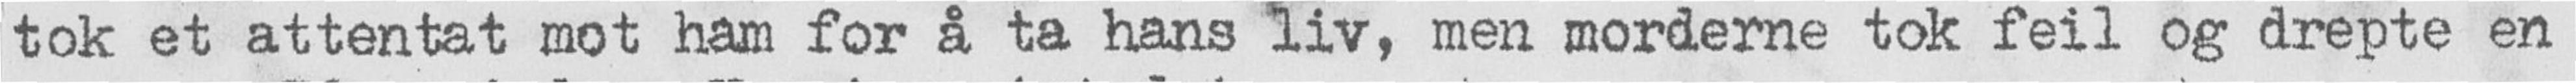tok et attentat mot ham for å ta hans liv, men morderne tok feil og drepte en
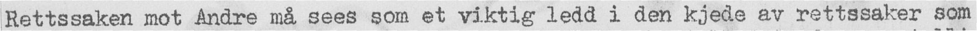Rettssaken mot Andre må sees som et viktig ledd i den kjede av rettssaker som
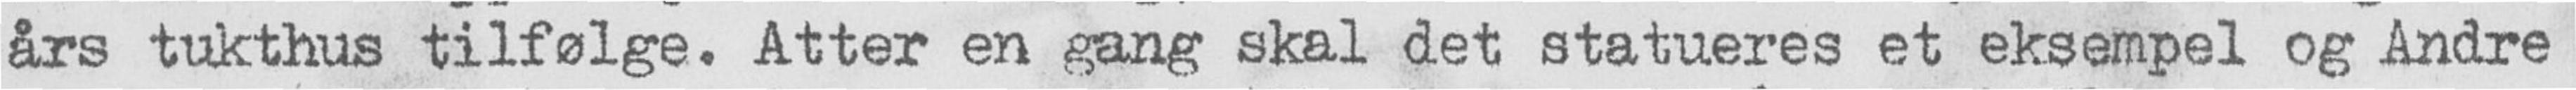års tukthus tilfølge. Atter en gang skal det statueres et eksempel og Andre
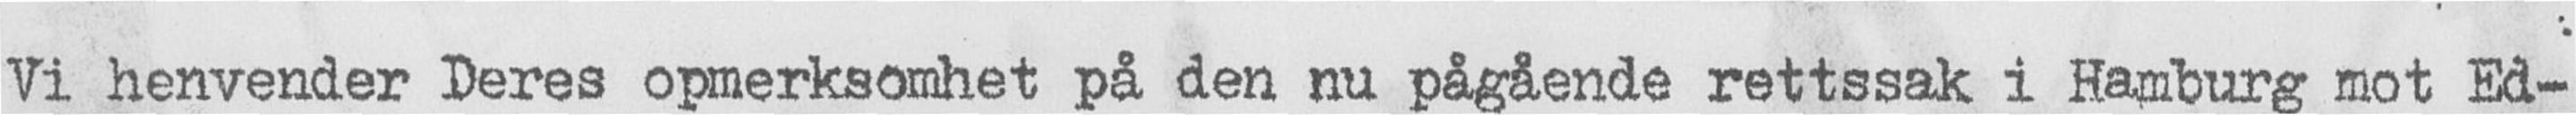Vi henvender Deres opmerksomhet på den nu pågående rettssak i Hamburg mot Ed-
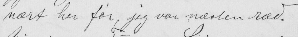vært her før, jeg var næsten ræd.
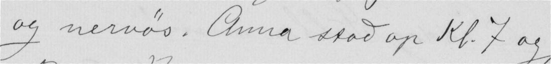og nervøs. Anna stod op Kl. 7 og
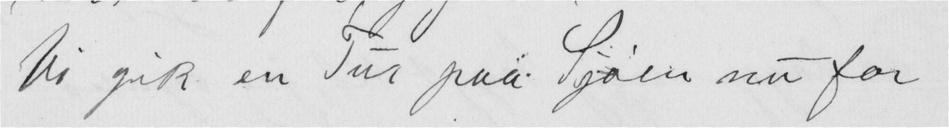Vi gik en Tur paa Sjøen nu for
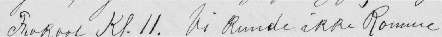Frokost Kl. 11. Vi Kunde ikke Komme
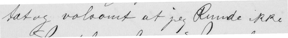tæt og voldsomt at jeg Kunde ikke
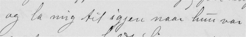og la mig til igjen naar hun var
